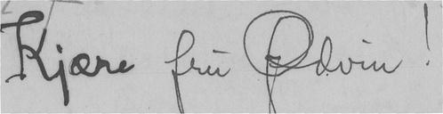Kjære fru Ødvin!
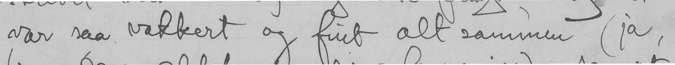var saa vakkert og fint alt sammen (ja,
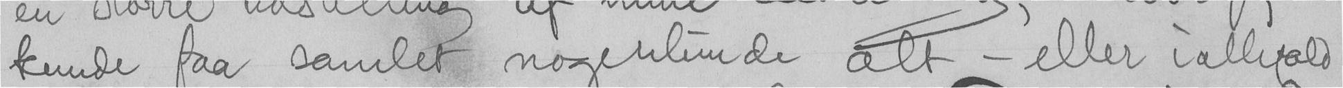kunde faa samlets nogenlunde alt - eller iallefald
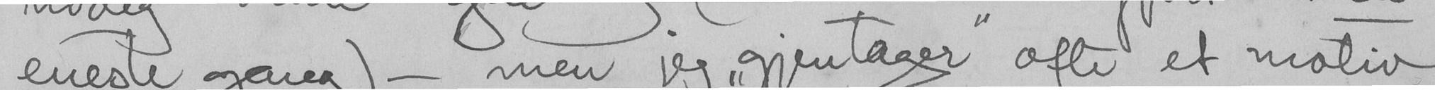eneste gang), - men jeg "gjentager" ofte et motiv
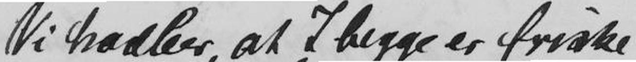Vi haaber, at I begge er friske
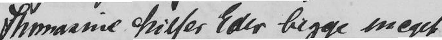Thomasine hilser Eder begge meget
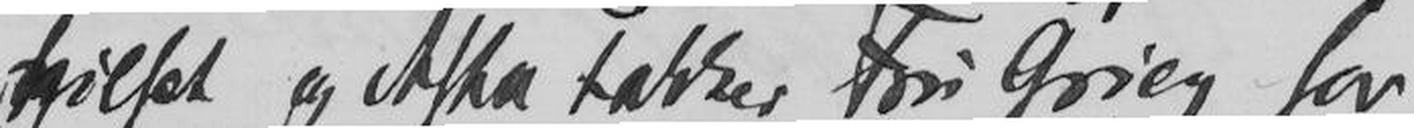hilset og Asta takker Fru Grieg for
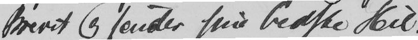Brevet og sender sine bedste Hil
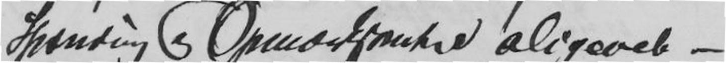Spænding og Opmærksomhed aligevel -
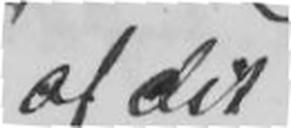af dit.
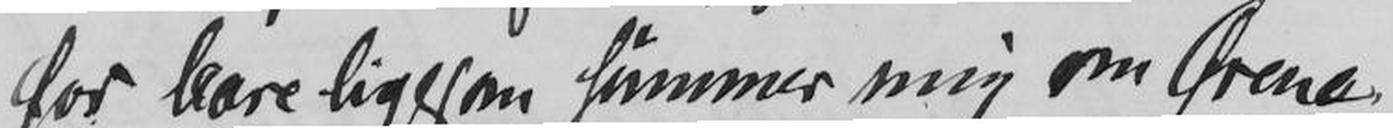for bare ligesom summer mig om Ørene,
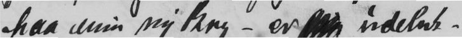paa min ny Bog - er udeluk-
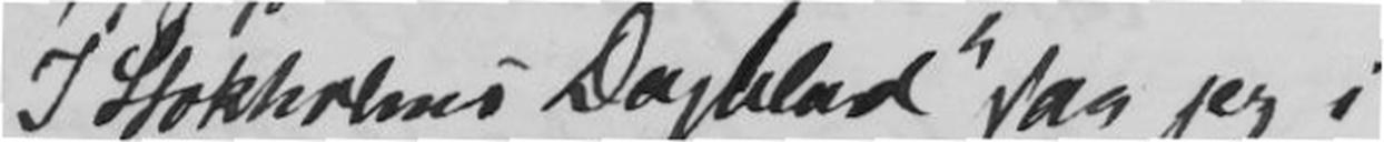I Stokholms Dagblad saa jeg i
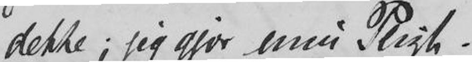dette; jeg gjør min Pligt.
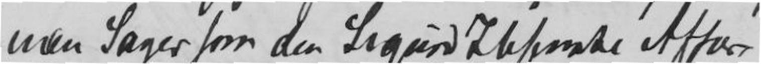men Sager som den Sigurd Ibsenske Affær
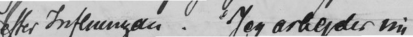efter Influensaen. Jeg arbejder nu
